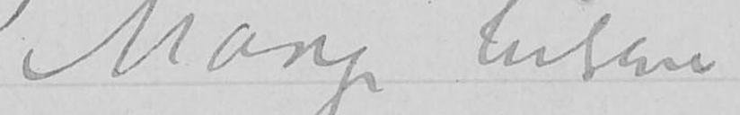Mange hilsener
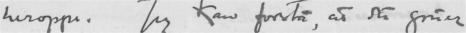heroppe. Jeg kan forstå, at du gruer
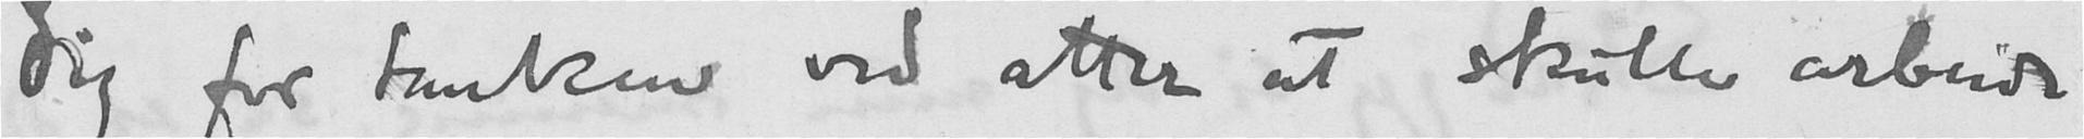dig for tanken ved atter at skulle arbeide
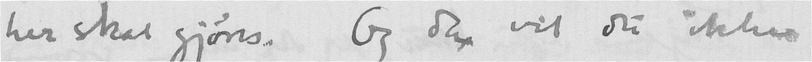her skal gjøres. Og da vil du ikke
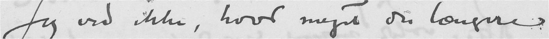Jeg ved ikke, hvor meget du længere
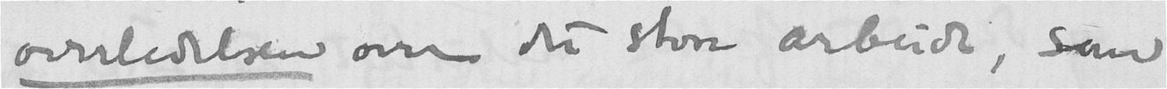overledelsen om det store arbeide, som
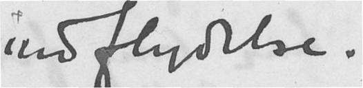indflydelse.
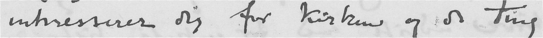interesserer dig for kirken og de ting
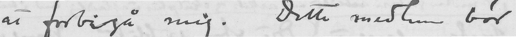at forbigå mig. Dette medlem bør
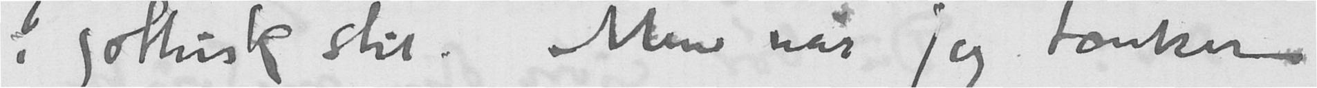i gothisk stil. Men når jeg tænker
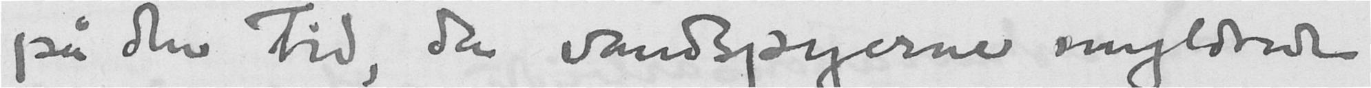på den tid, da vandspyerne myldrede
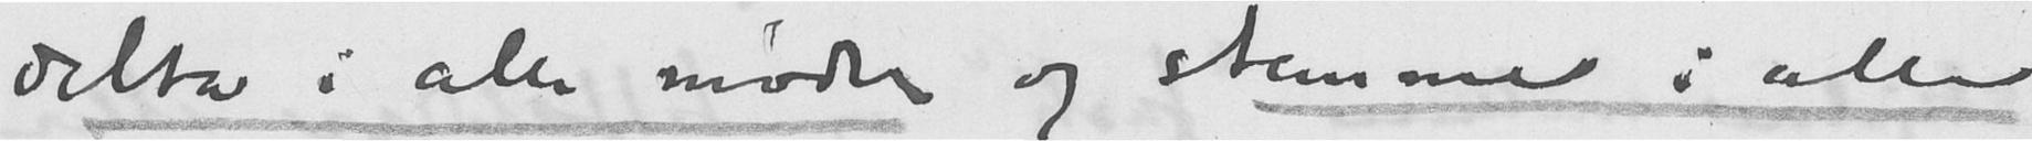delta i alle møder og stemme i alle
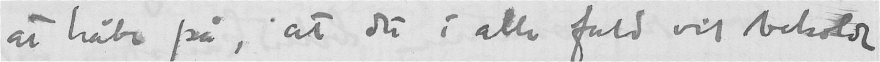at håbe på, at du i alle fald vil beholde
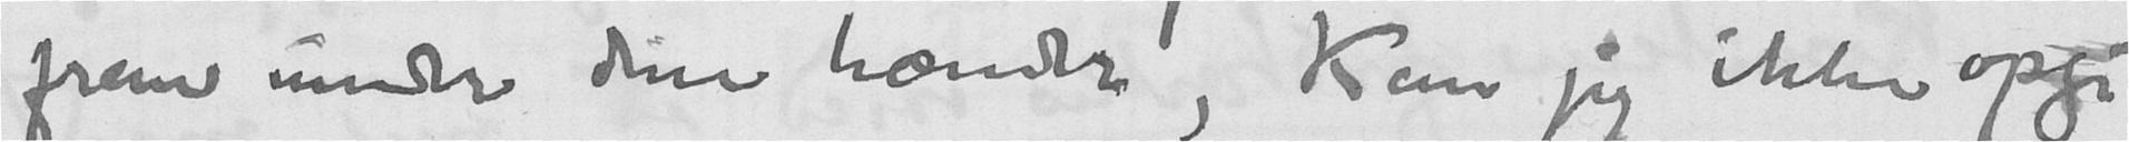frem under dine hænder, kan jeg ikke opgi
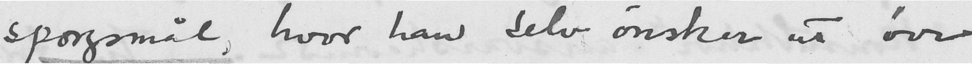spørgsmål, hvor han selv ønsker at øve
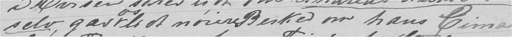selv, gav os lidt nøiere Besked om hans Cima
